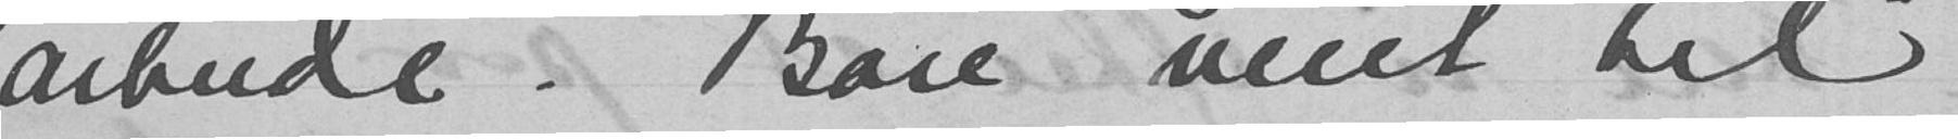arbeide. Bare vent til
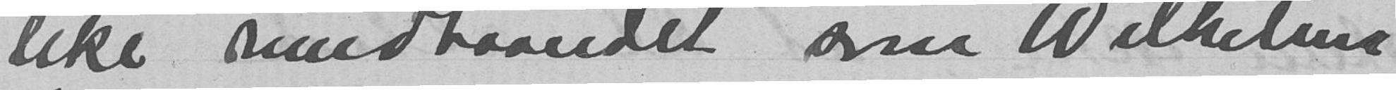like rundhaandet som Wilhelm
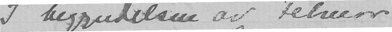I begyndelsen av Februar
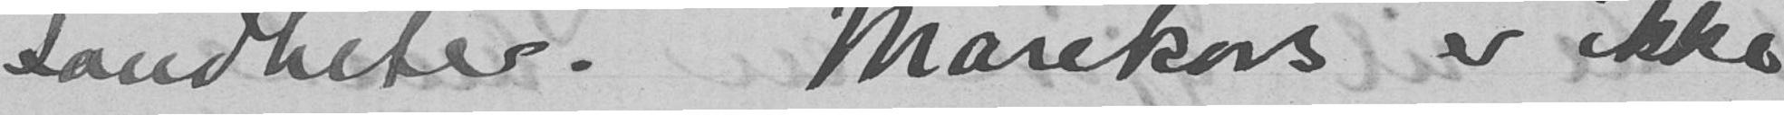sandheter. Marekors er ikke
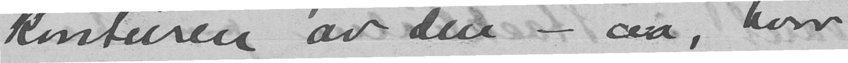konturen av den - aa, hvor
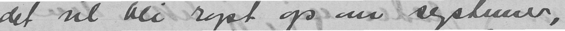det vil bli ropt op om systemer,
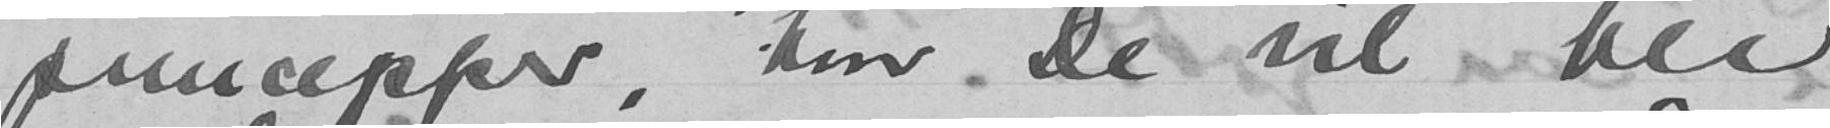principper, hvor De vil bli
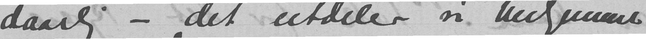daarlig - det utdeler vi herhjemme
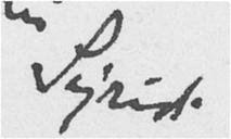Sigrid.
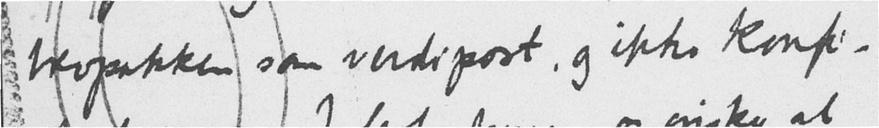brevpakken som verdipost, og ikke konfi-
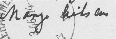Mange hilsener
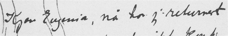Kjære Eugenia, nå har jeg returnert
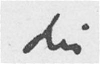din
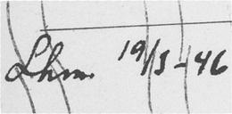Lhm. 19/3-46
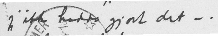jeg ikke hadde gjort det –.
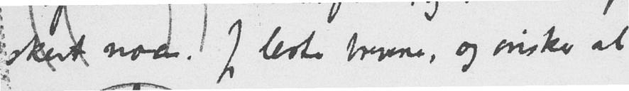skert noen. Jeg leste brevene, og ønsker at
